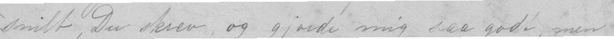snilt, Du skrev og gjorde mig saa godt, men
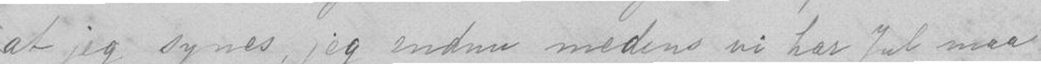at jeg synes, jeg endnu medens ni har Jul maa i
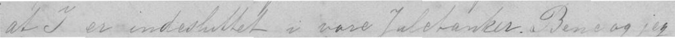at I er indesluttet i vore Juletanker. Bene og jeg
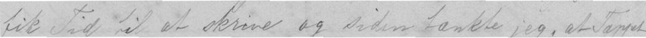fik Tid til at skrive og siden tænkte jeg, at Tæppet
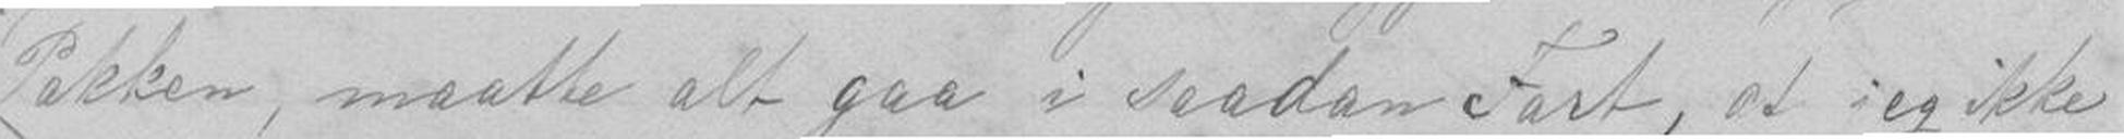Pakken, maatte alt gaa i saadan Fart, at jeg ikke
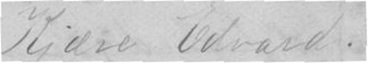Kjære Edvart!
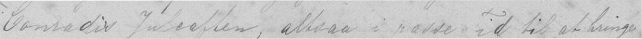Conradis Juleaften, altsaa i passe Tid til at bringe
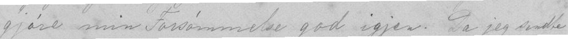gjøre min Forsømmelse god igjen. Da jeg sendte
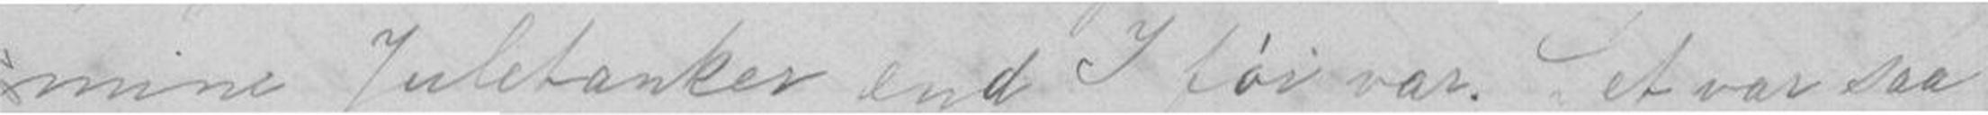mine Juletanker end I før var. Det var saa
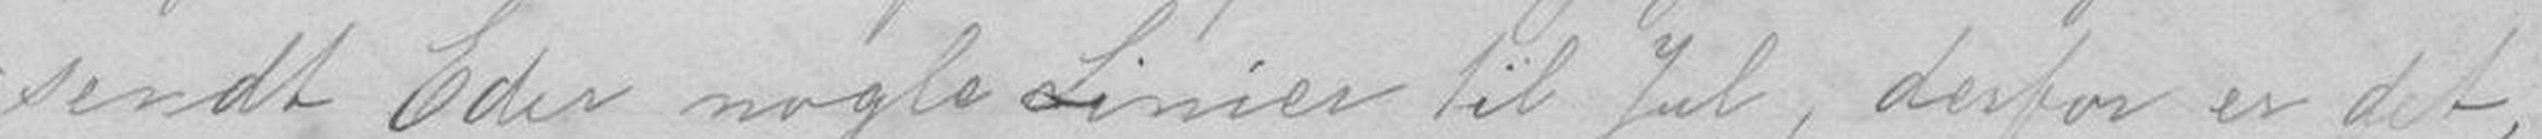sendt Eder nogle Linier til Jul, derfor er det,
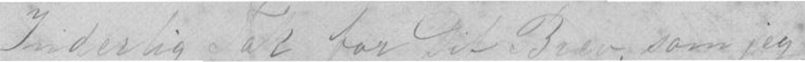Inderlig Tak for Dit Brev som jeg
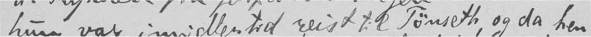hun var imidlertid reist til Tønseth, og da hen-
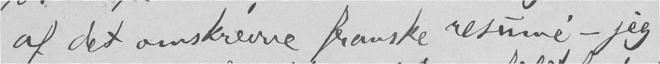af det omskrevne franske resumé - jeg
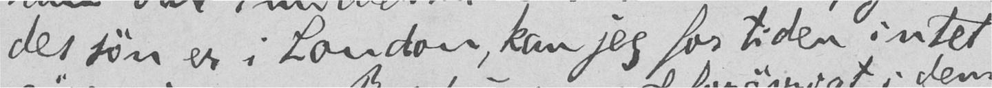des søn er i London, kan jeg for tiden intet
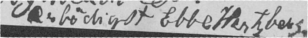Ærbødigst Ebbe Hertzberg.
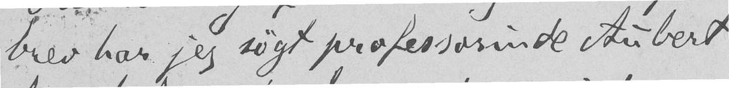brev har jeg søgt professorinde Aubert
